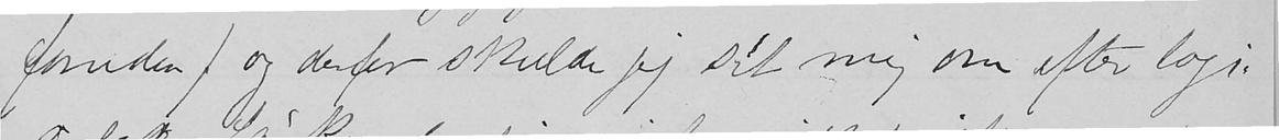foruden) og derfor skulde jeg sét mig om efter logi,
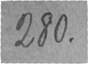280.
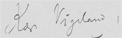Kære Vigeland,
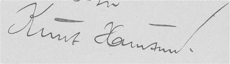Knut Hamsun.
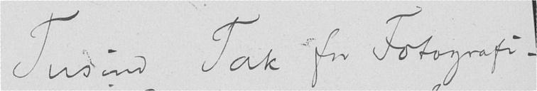Tusind Tak for Fotografi-
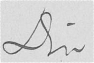Din
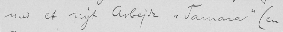med et nyt Arbejde "Tamara" (en
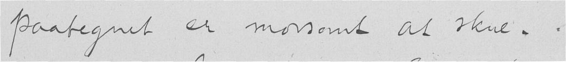paategnet er morsomt at skue.
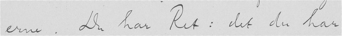erne. Du har Ret: det du har
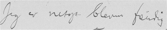Jeg er netop bleven ferdig
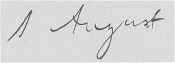1 August
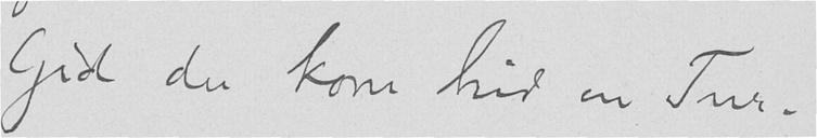Gid du kom hid en Tur.
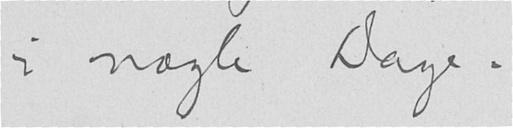i nogle Dage.
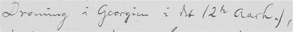Dronning i Georgia i det 12te Aarh.),
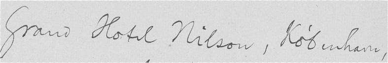Grand Hotel Nilson, København,
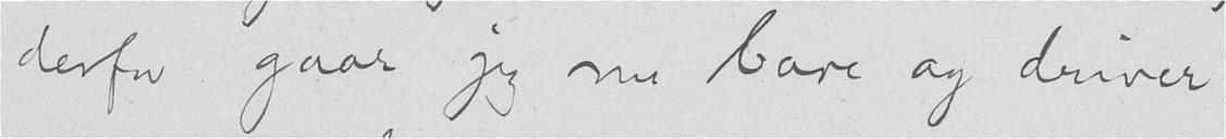derfor gaar jeg nu bare og driver
